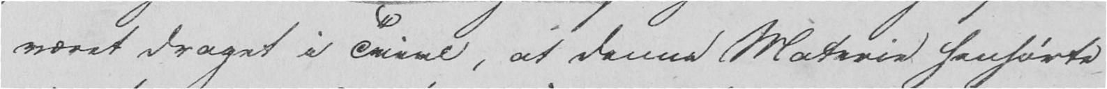været draget i Tvivl, at denne Materie henhørte
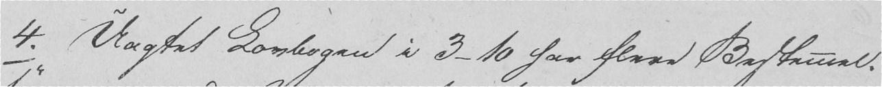4. Uagtet Lovbogen i 3-10 har flere Bestem̄el-
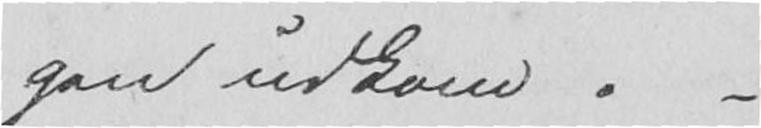gen udkom. -
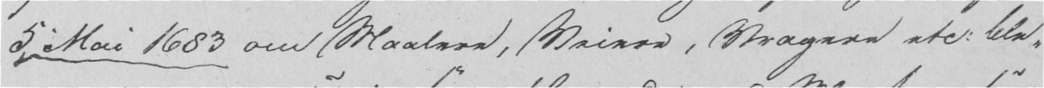5 Mai 1683 om Maalere, Veiere, Vragere etc. ble-
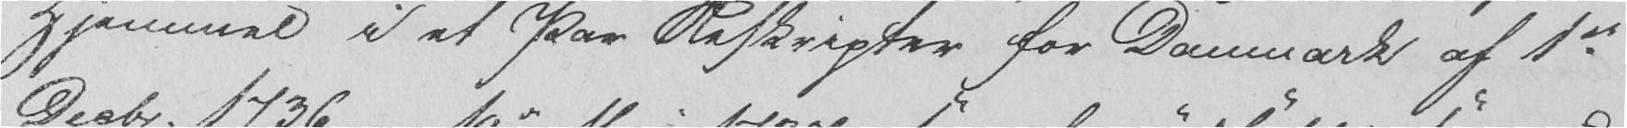Hjemmel i et Par Reskripter for Danmark af 1de
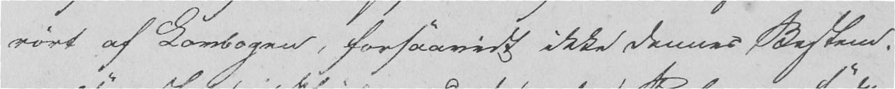rørt af Lovbogen, forsaavidt ikke dennes Bestem-
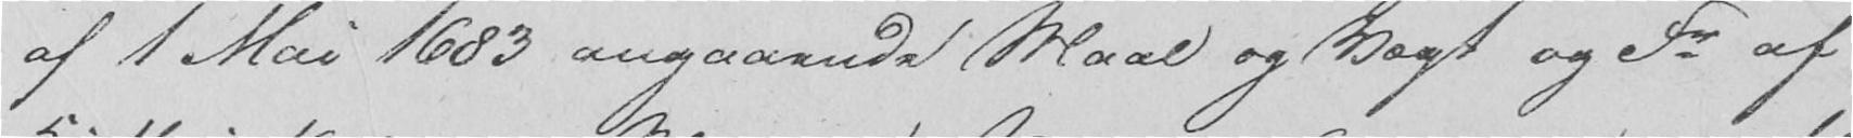af 1 Mai 1683 angaaende Maal og Vægt og Fr af
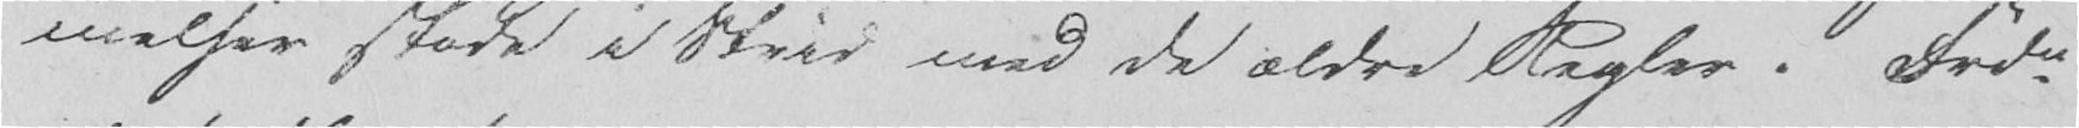melser stode i Strid med de ældre Regler. Frdn
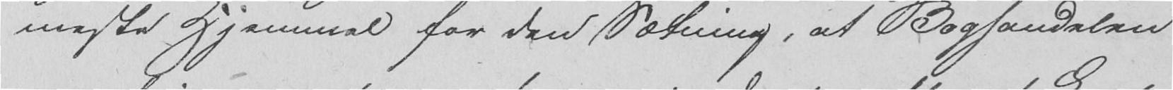meste Hjemmel for den Sætning, at Boghandelen
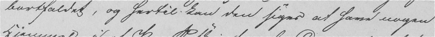bortfaldet, og hertil kan den siges at have nogen
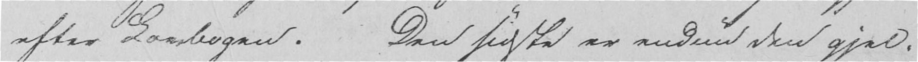efter Lovbogen. Den sidste er endnu den gjel-
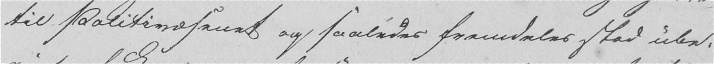til Politivæsenet og saaledes fremdeles stod ube-
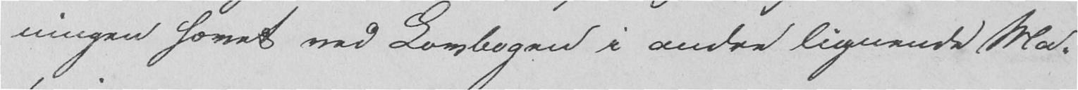ningen hævet ved Lovbogen i andre lignende Ma-
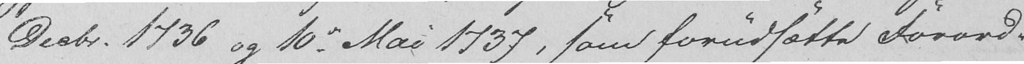Decbr. 1736 og 10. Mai 1737, som forudsætte Forord-
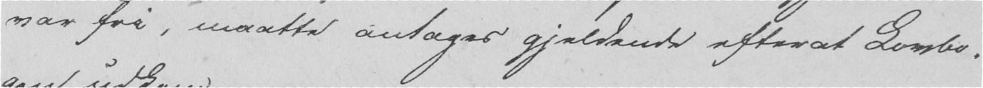var fri, maatte antages gjeldende efterat Lovbo-
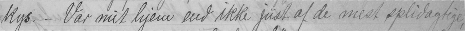kys. - Var mit hjem end ikke just af de mest splidagtige,
